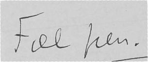Fæl pen.
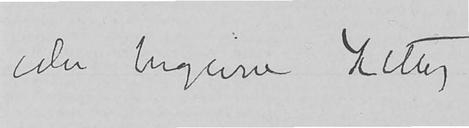eders hengivne Kitty.
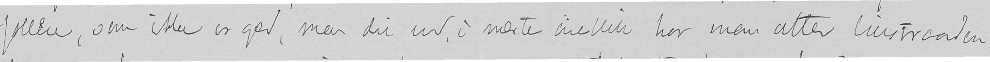følelse, som ikke er god, men du ved, i næste øieblik har man atter livstraaden
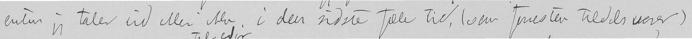enten jeg taler ud eller ikke, i den sidste fæle tid, (som forresten tildels varer)
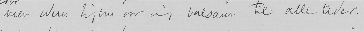men eders hjem var mig balsam til alle tider.
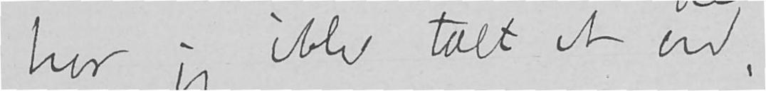har jeg ikke talt et ord,
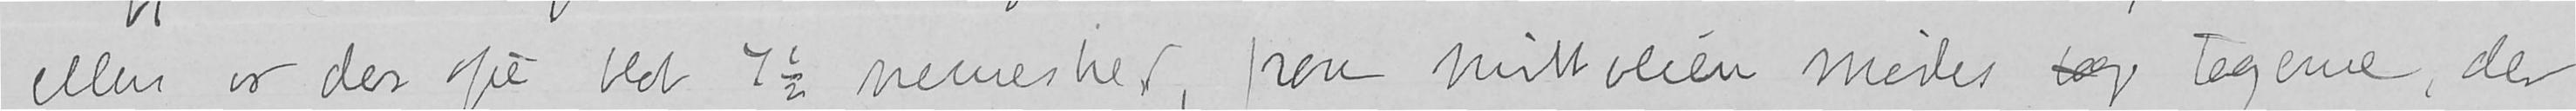ellers er der ofte blot 7½ mennesker, paa midtveien møder tæg togerne, der
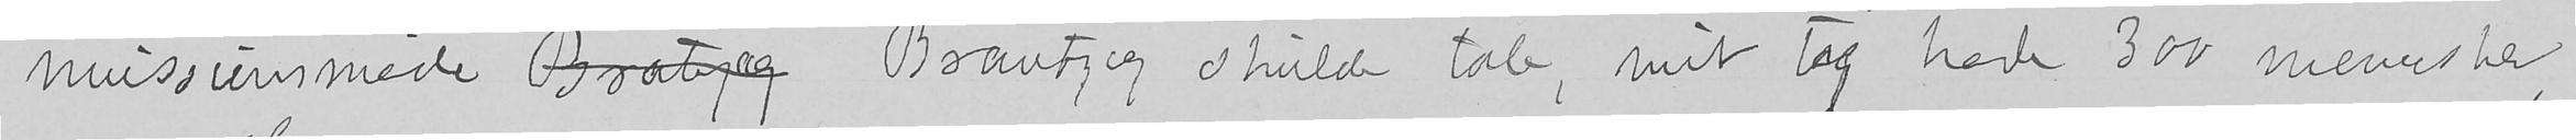museumsmøde Bratzeg Brantzeg skulde tale, mit tog havde 300 mennesker,
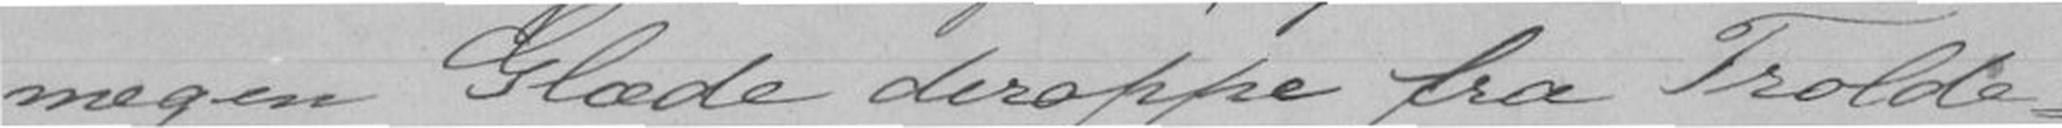megen Glæde deroppe fra Trolde
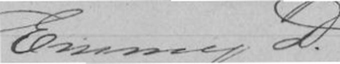Emmy D.
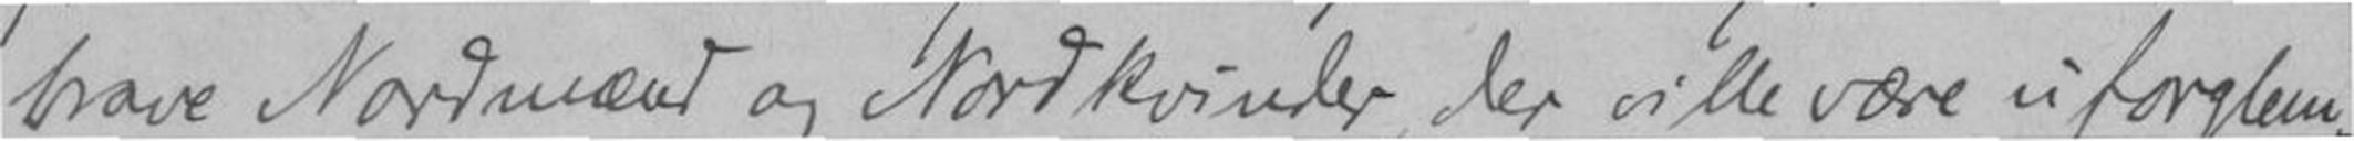brave Nordmænd og Nordkvinder, der ville være uforglem-
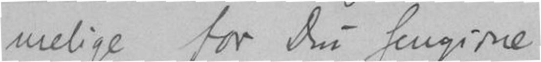melige for Din hengivne
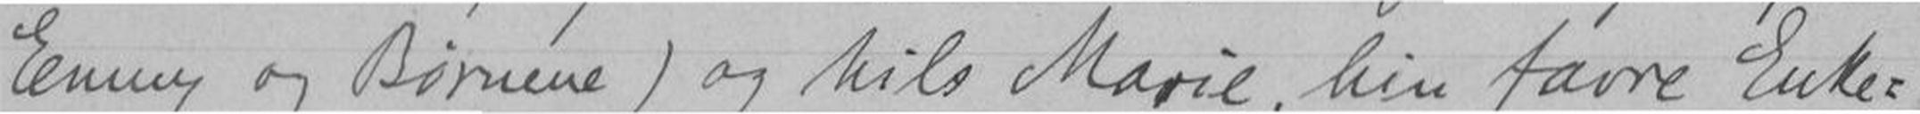Emmy og Børnene) og hils Marie. Min favre Enke-
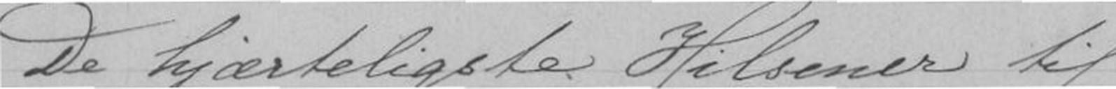De hjærteligste Hilsener til
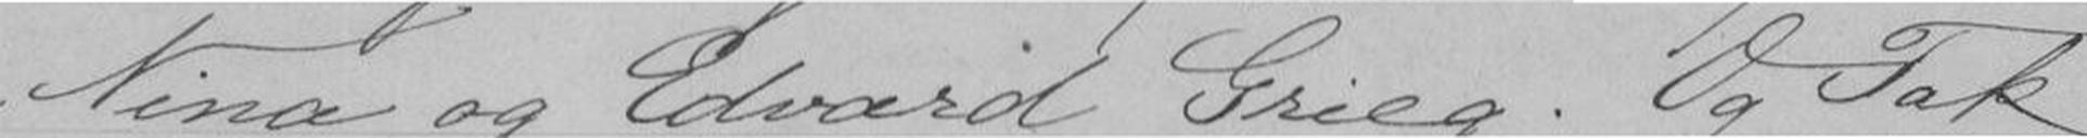Nina og Edvard Grieg. Og Tak
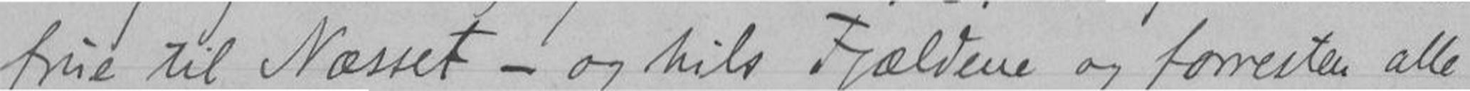frue til Næsset - og hils Fjældene og forresten alle
